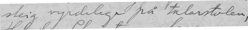steig vyrdelege på talarstolen,
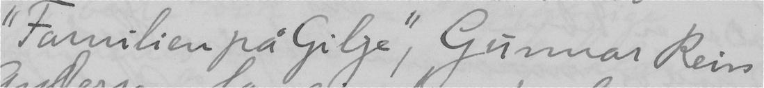"Familien på Gilje," Gunnar Reiss
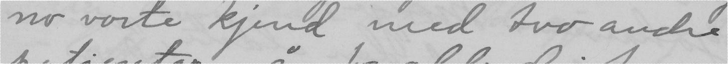no vorte kjend med tvo andre
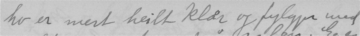ho er mest heilt klår og fylgjer med
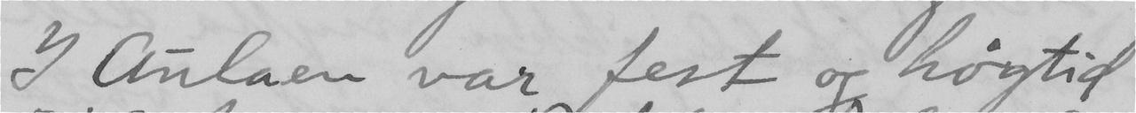I Aulaen var fest og högtid
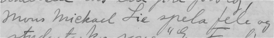Mons Mickael Lie spela fele og
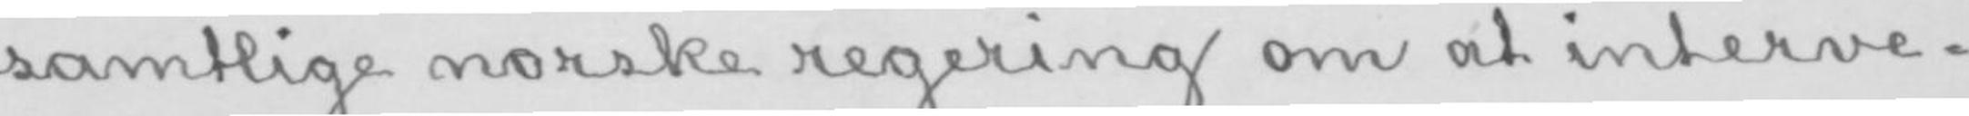samtlige norske regering om at interve-
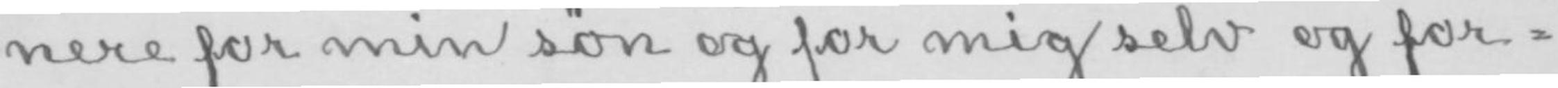nere for min søn og for mig selv og for-
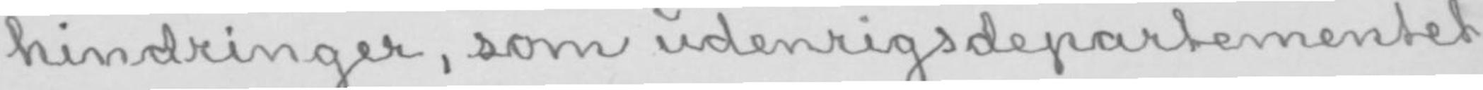hindringer, som udenrigsdepartementet
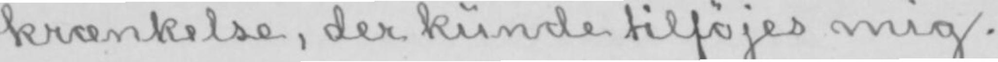krænkelse, der kunde tilføjes mig.
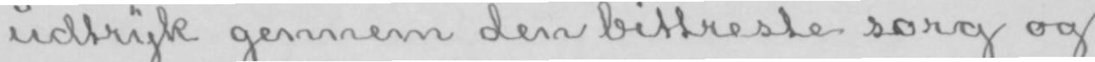udtryk gennem den bittreste sorg og
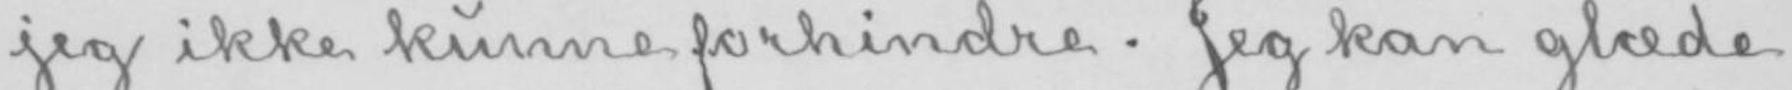jeg ikke kunne forhindre. Jeg kan glæde
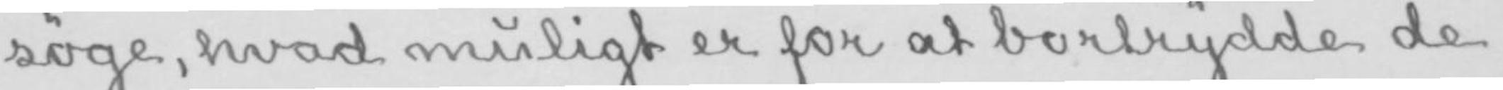søge, hvad muligt er for at bortrydde de
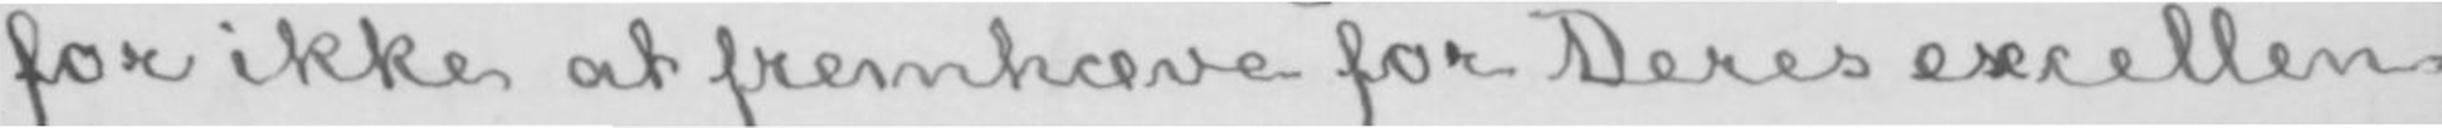for ikke at fremhæve for Deres excellen-
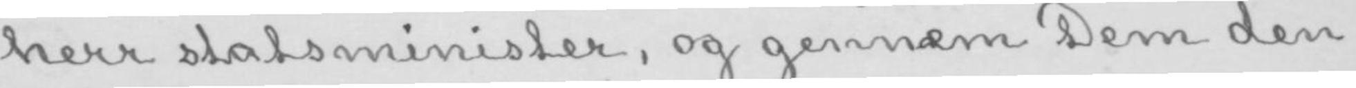herr statsminister, og gennem Dem den
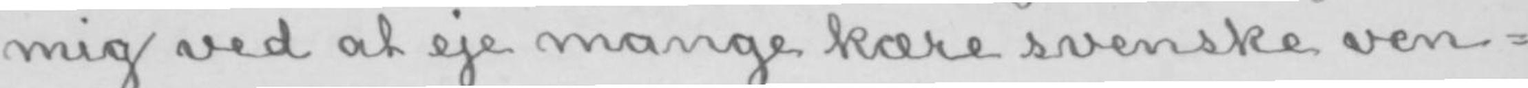mig ved at eje mange kære svenske ven-
